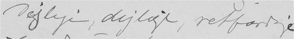Dejlige, dejlige, retfærdige
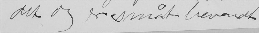det dog er småt bevendt
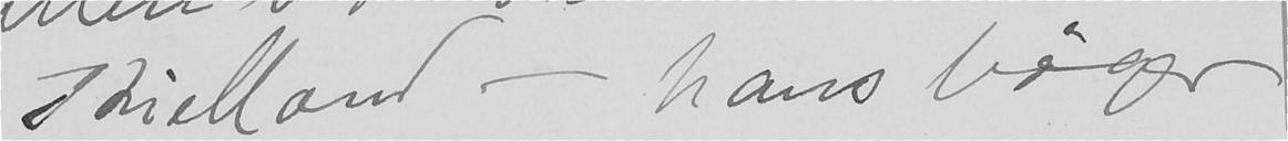Kielland - hans bøger
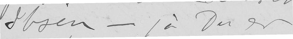Ibsen - ja Du er
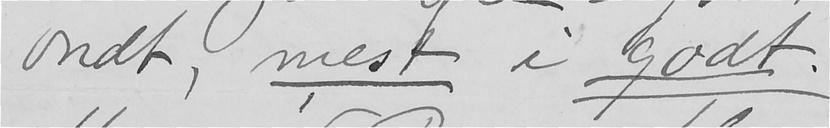ondt, mest i godt.
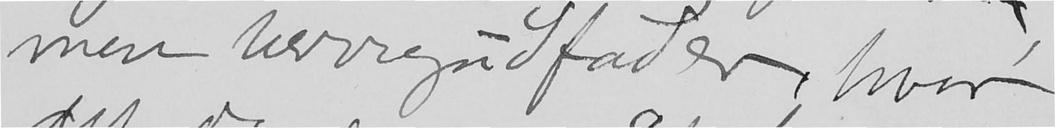men herregudfader, hvor
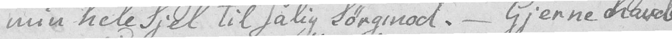min hele Sjel til salig Sørgmod. – Gjerne havde
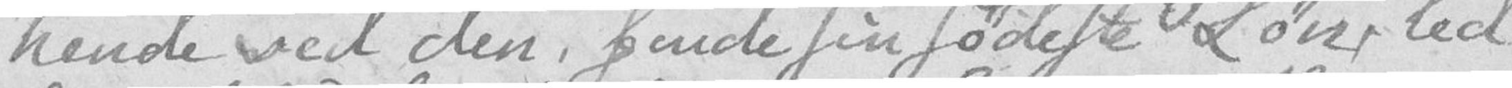hende ved den, finde sin sødeste Løn, led
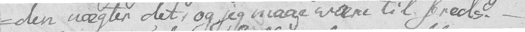-den nægter det, og jeg maae være til freds. –
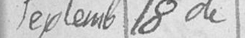September 18de
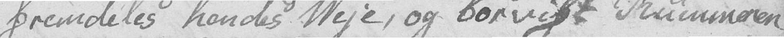fremdeles hendes Veje, og borvist Kummeren
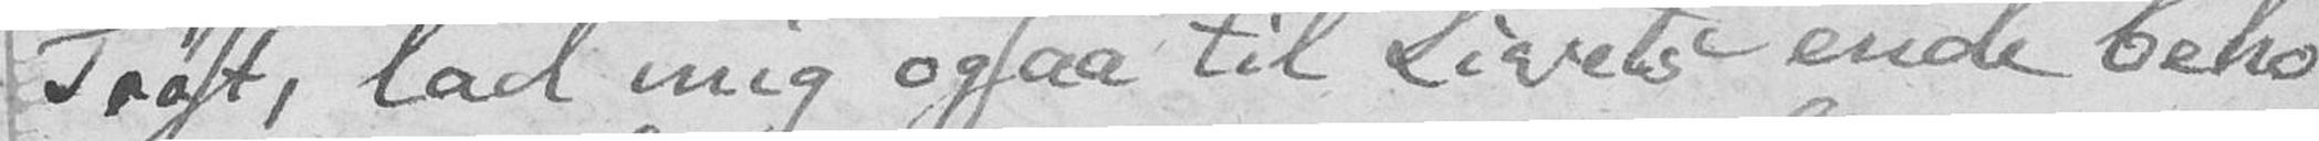Trøst, lad mig ogsaa til Livets ende beho
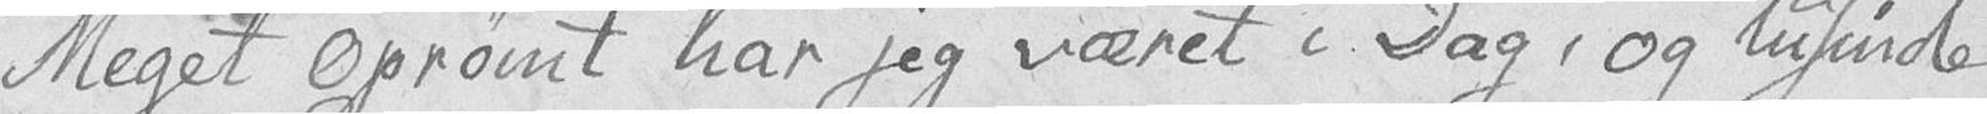Meget Oprømt har jeg været i Dag, og tusinde
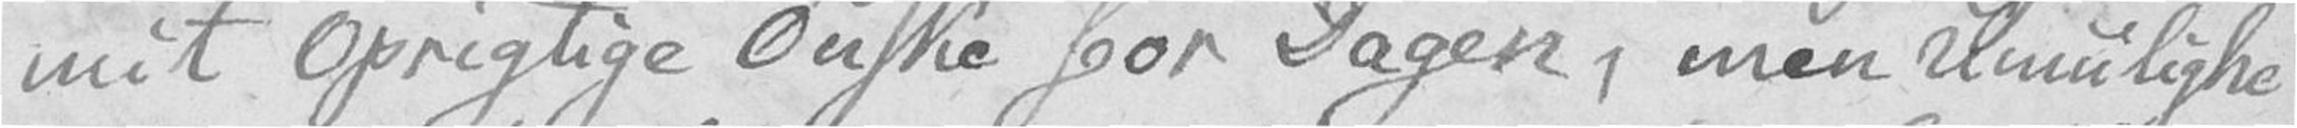mit Oprigtige Ønske for Dagen, men Umulighe
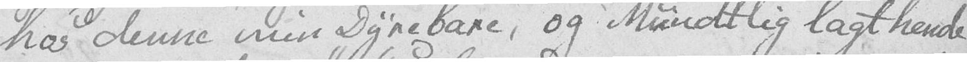hos denne min Dyrebare, og Mundtlig lagt hende
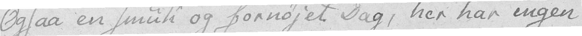Ogsaa en smuk og fornøjet Dag, her har ingen
